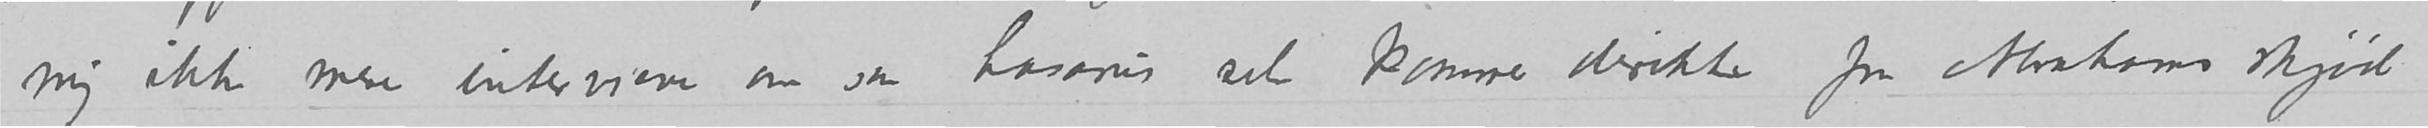mig ikke mere intervieve om saa Lasarus selv kommer direkte fra Abrahams skjød
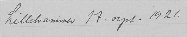Lillehammer 17. sept. 1921
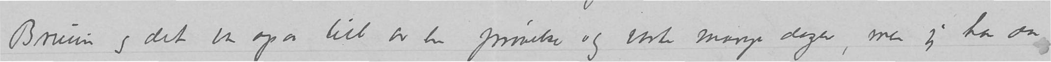Bruun og det var ogsaa litt av en prøvelse og varte mange dager, men jeg har da
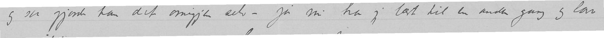og saa gjorde han det om igjen selv – ja nu har jeg lært til en anden gang og lar
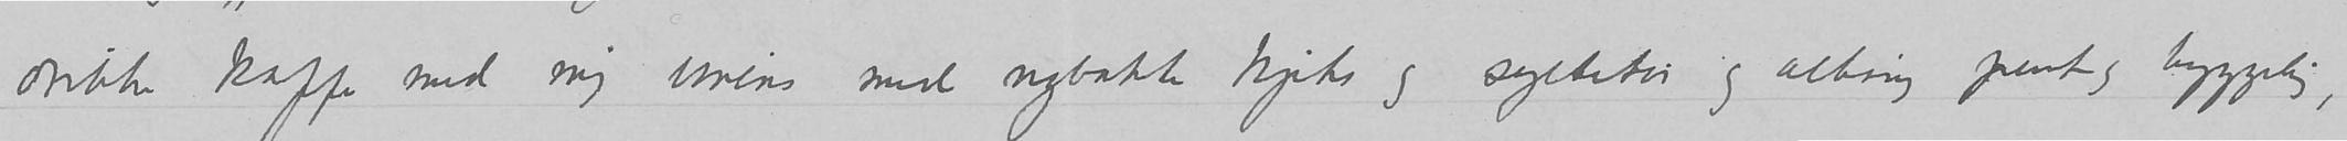drikke kaffe med mig imens med nybakte kjeks og syltetøi og alting pent og hyggelig,
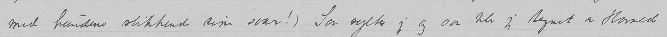med hundene slikkende sine saar! Saa sylter jeg og saa blev jeg tegnet av Harald
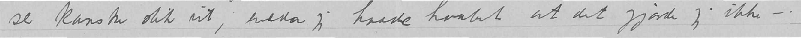ser kanske slik ut, endda jeg hadde haabet at det gjorde jeg ikke –?
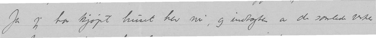Ja jeg har kjøpt huset her nu, og indtægten av de samlede verker
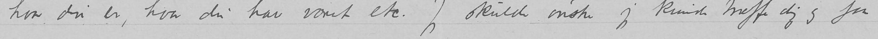hvor du er, hvor du har været etc. Jeg skulde ønske jeg kunde træffe dig og faa
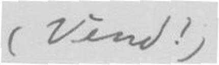(Vend!)
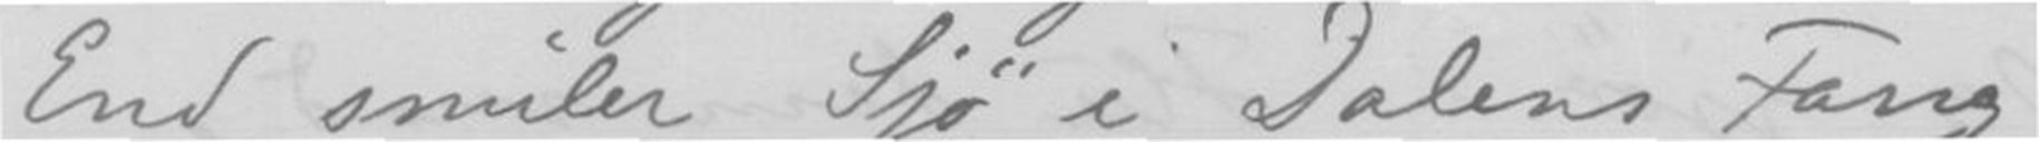End smiler Sjø i Dalens Fang,
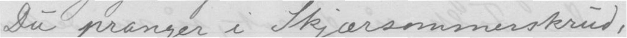Du pranger i Skjærsommerskrud,
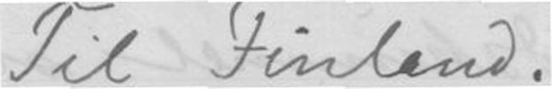Til Finland.
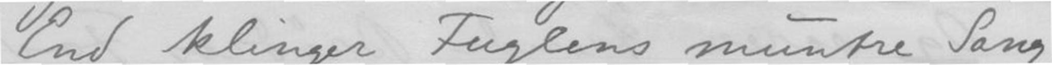End klinger Fuglens muntre Sang,
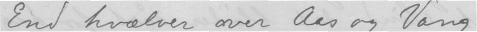End hvælver over Aas og Vang
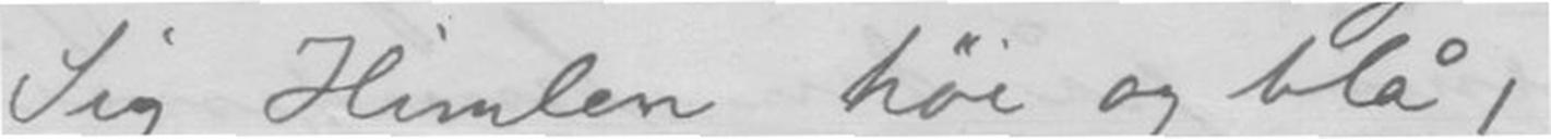Sig Himlen høi og blå,
